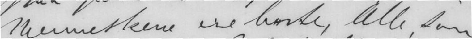Menneskene ere borte, Alle, som
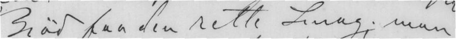Brød faa den rette Smag; man
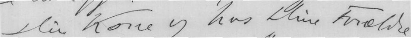Din Kone og hos Dine Forældre
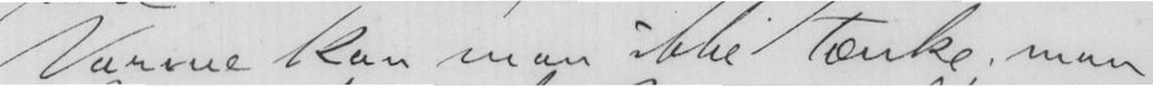Varme kan man ikke tænke; man
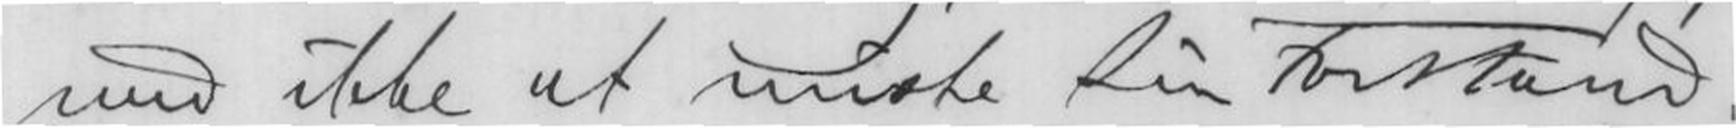ved ikke at miste sin Forstand.
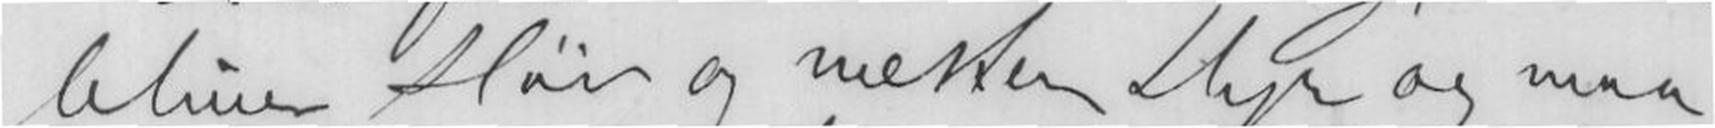bliver sløv og næsten Dyr og maa
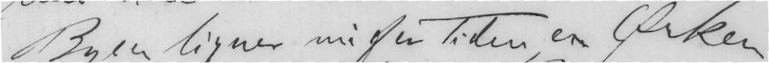Byen ligner nu for Tiden en Ørken,
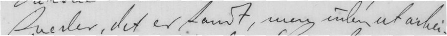Sveder, det er sandt, men uden at arbei-
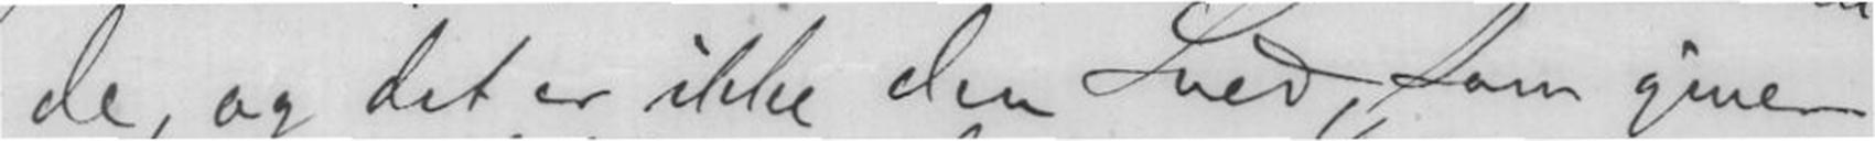de, og det er ikke den Sved, som giver
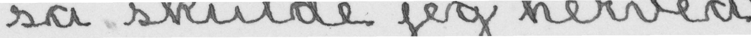så skulde jeg herved
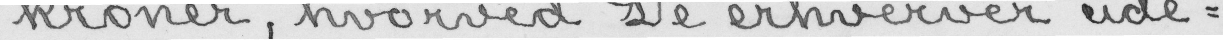kroner, hvorved De erhverver ude-
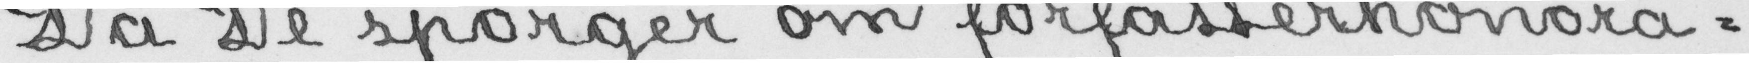Da De spørger om forfatterhonora-
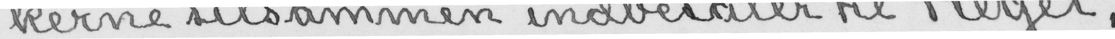kerne tilsammen indbetaler til Hegel,
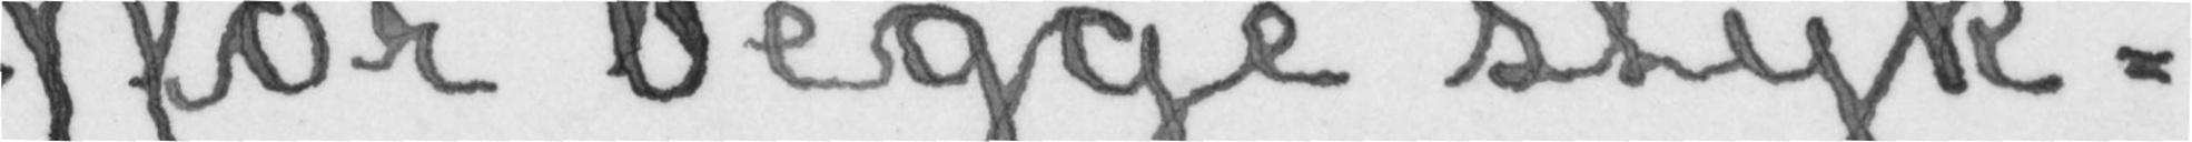for begge styk-
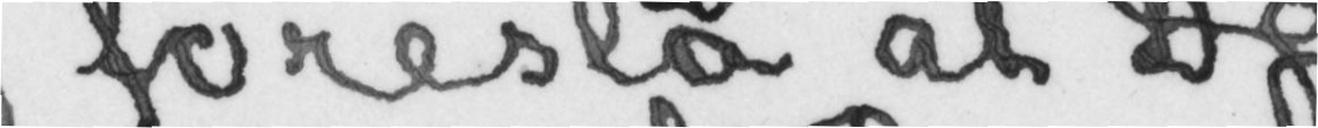foreslå at De
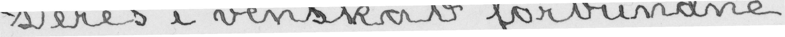Deres i venskab forbundne
